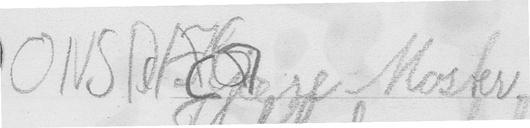ONSDAG
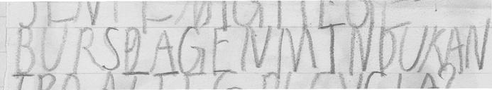BURSDAGEN MIN DU KAN
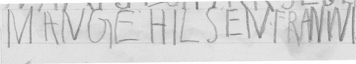MANGE HILSEN FRANNI.
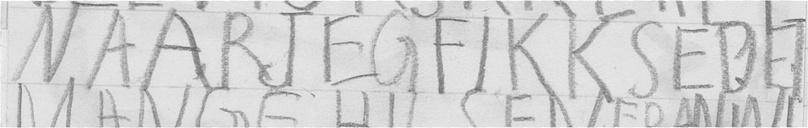NAAR JEG FIK SE DET
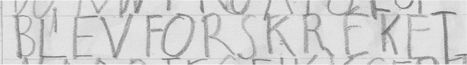BLEV FORSKREKET
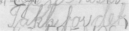Takk for det
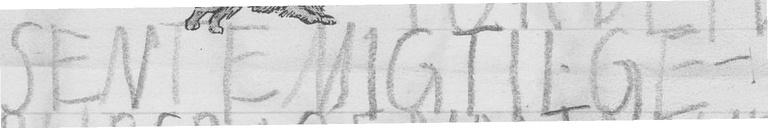SENTE MIG TIL GE-
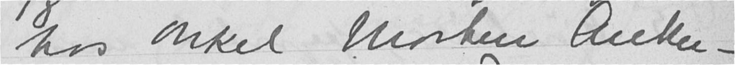hos onkel Morten Anker -
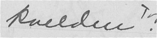kvelden.
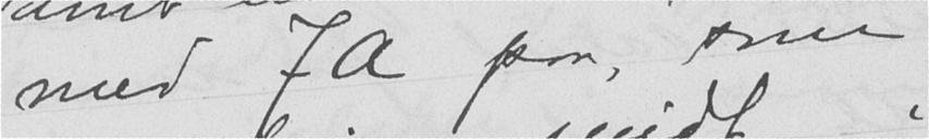med JA paa, som
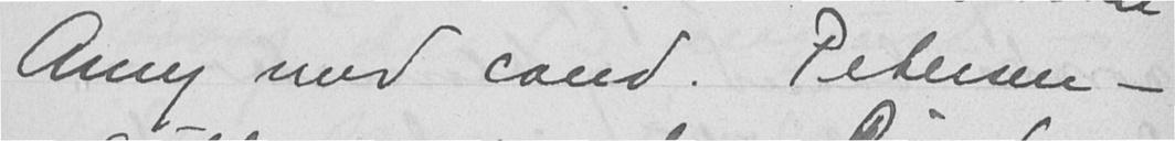Amy med cand. Petersen -
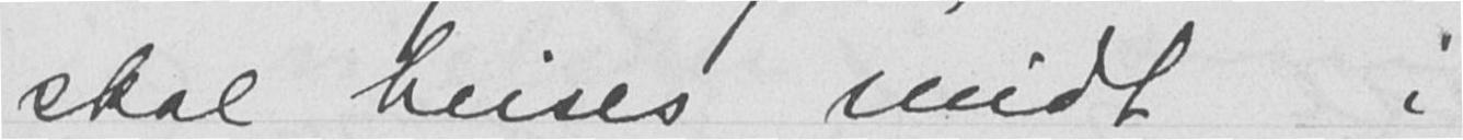skal heises midt i
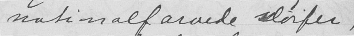nationalfarvede sløifer,
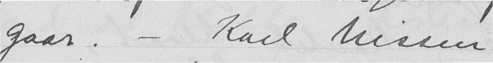gaar. - Karl Nissen
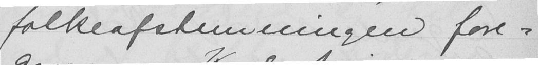folkeafstemningen fore-
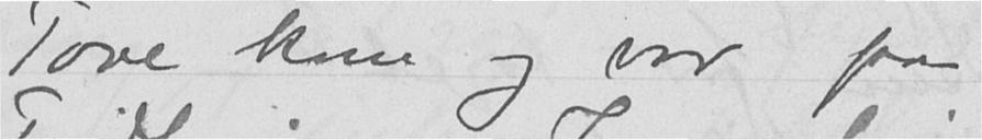Tove kom og var paa
